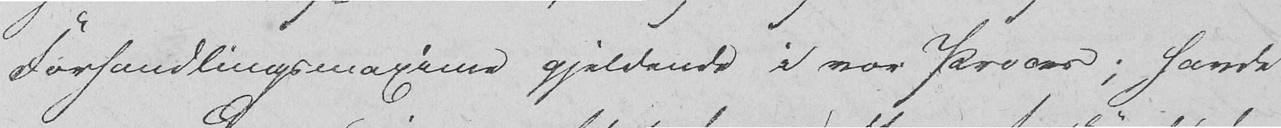Forhandlingsmaxime gjeldende i vor Proces; havde
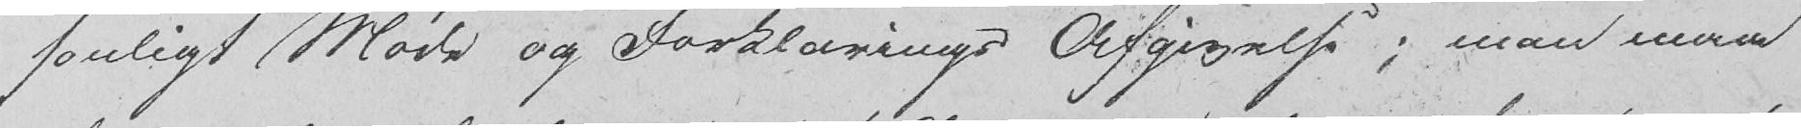sonligt Møde og Forklarings Afgivelse; man maa
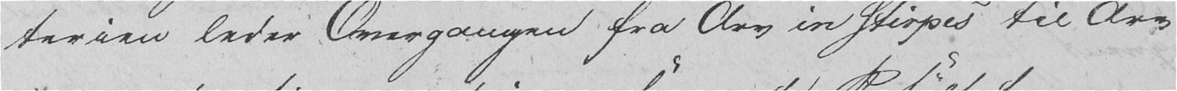terien leder Overgangen fra Arv in stirpes til Arv
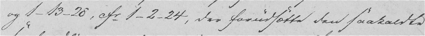og 1-13-28, cfr 1-2-24, der forudsætte den saakaldte
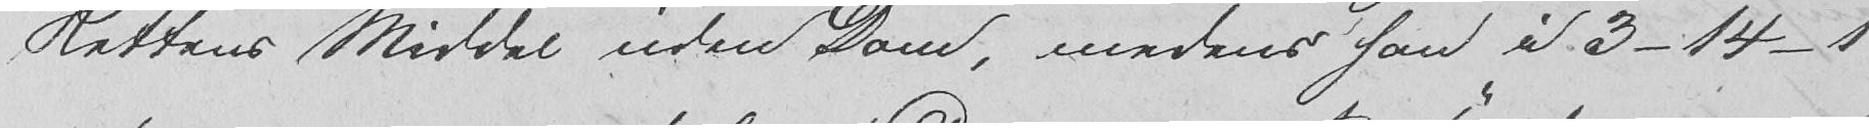Rettens Middel uden Dom, medens han i 3-14-1
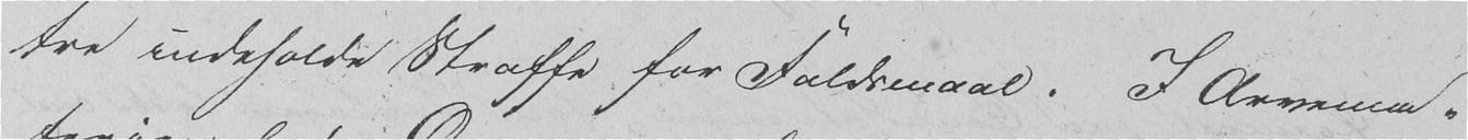tre indeholde Straffe for Faldsmaal. I Arvema-
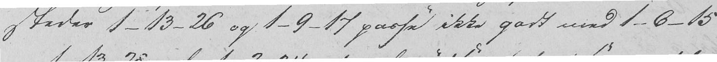steder 1-13-26 og 1-9-17 passe ikke godt med 1-6-15
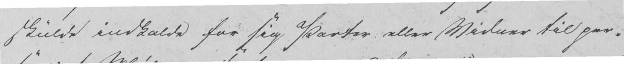skulde indkalde for sig Parter eller Vidner til per-
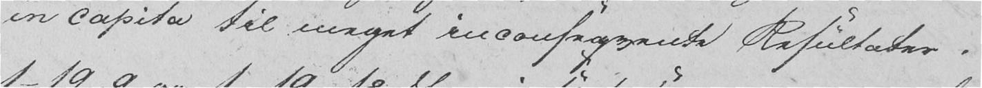in capita til meget inconseqvente Resultater.
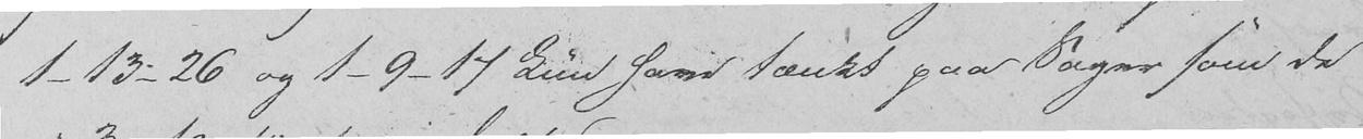1-13-26 og 1-9-17 kun have tænkt paa Sager som de
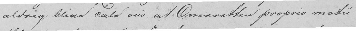aldrig blive Tale om at Overretten proprio motu
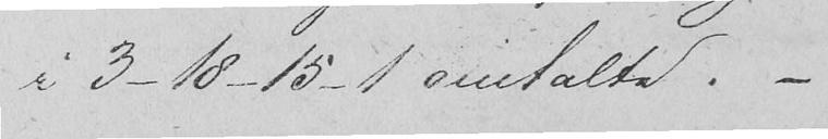i 3-18-15-1 omtalte. -
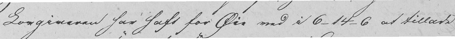Lovgiveren har haft for Øie ved i 6-14-6 at tillade
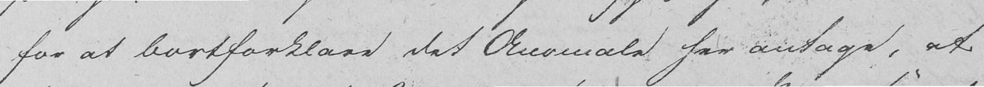for at bortforklare det Anomale her antage, at
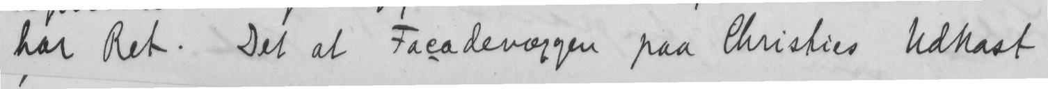har Ret. Det at Facadevæggen paa Cristies Udkast
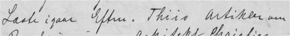Læste igaar Eften. Thiis Artikler om
